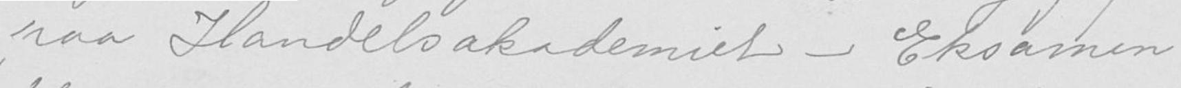paa Handelsakademiet - Eksamen
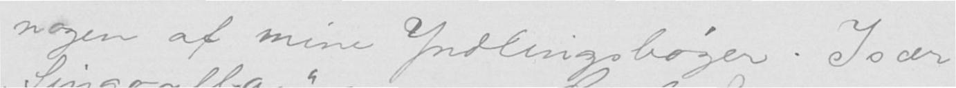nogen af mine Yndlingsbøger. Især
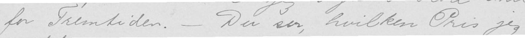for Fremtiden. - Du ser, hvilken Pris jeg
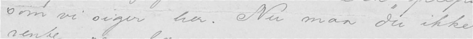som vi siger her. Nu maa du ikke
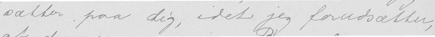sætter paa dig, idet jeg forudsætter,
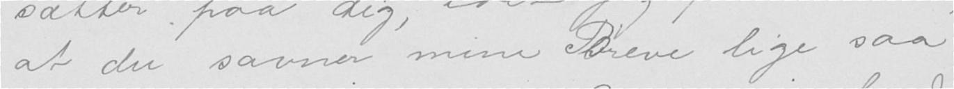at du savner mine Breve lige saa
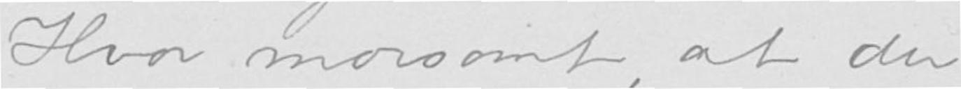Hvor morsomt, at du
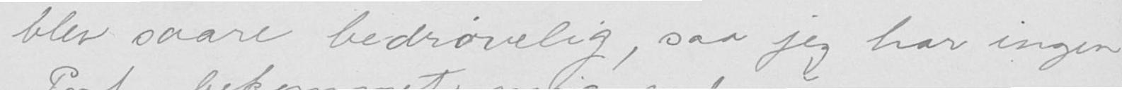blev saare bedrøvelig, saa jeg har ingen
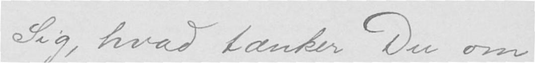Sig, hvad tænker Du om
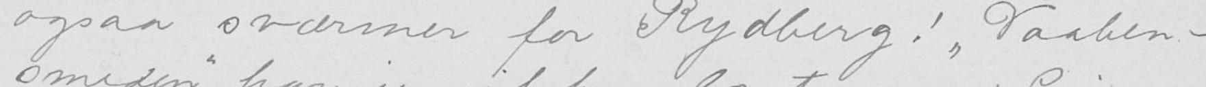ogsaa sværmer for Rydberg! "Vaaben-
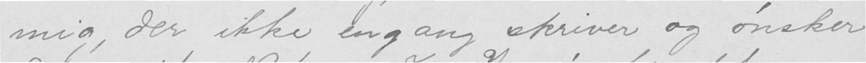mig, der ikke engang skriver og ønsker
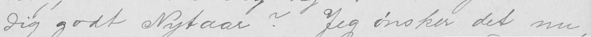dig godt Nytaar? Jeg ønsker det nu,
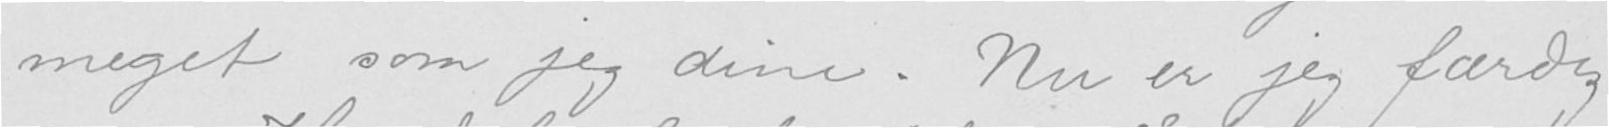meget som jeg dine. Nu er jeg færdig
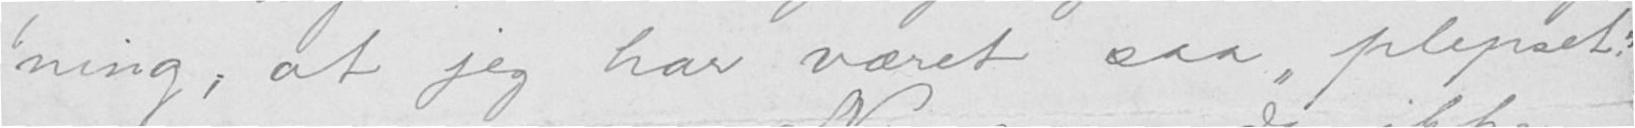ning, at jeg har været saa "plepset"
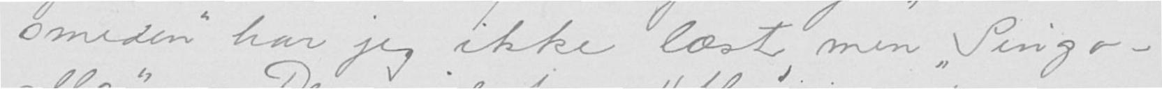smeden" har jeg ikke læst, men "Singo-
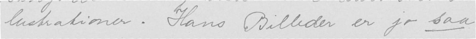lustrationer. Hans Billeder er jo saa
